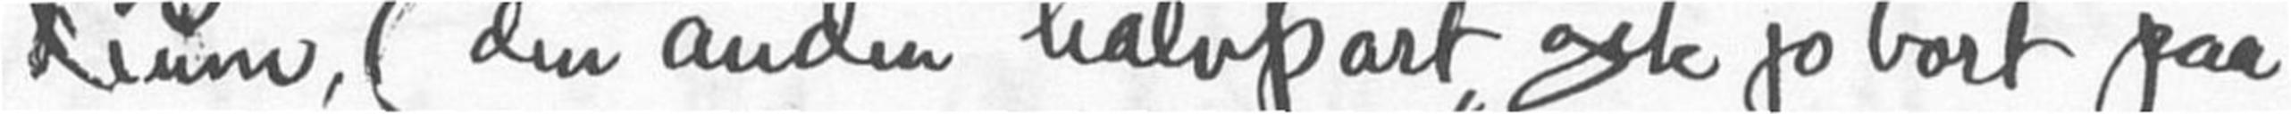dium, (den anden halvpart "gik jo bort" paa
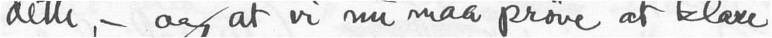dette, - og at vi nu maa prøve at klare
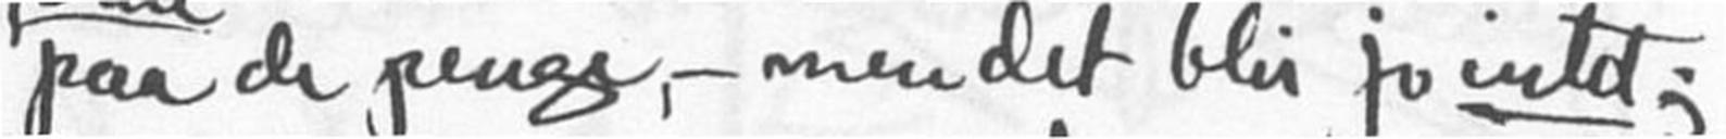paa de penge, men det blir jo intet;
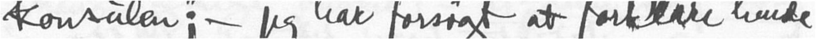konsulen"; - jeg har forsøgt at forklare hende
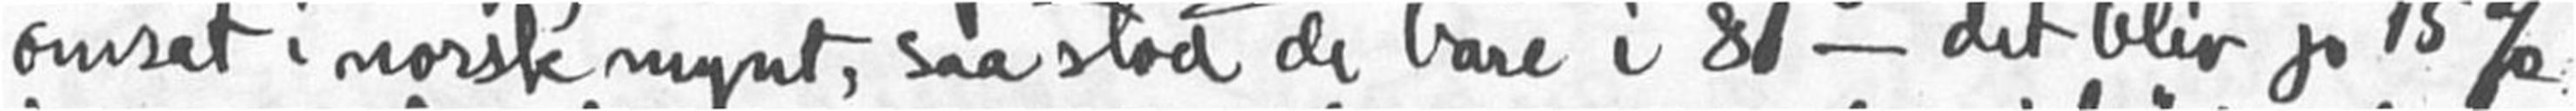omsat i norsk mynt, saa stod de bare i 81 - det blir jo 15 %
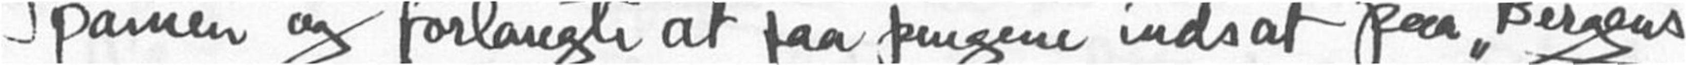Spanien og forlangte at faa pengene indsat paa "Bergens
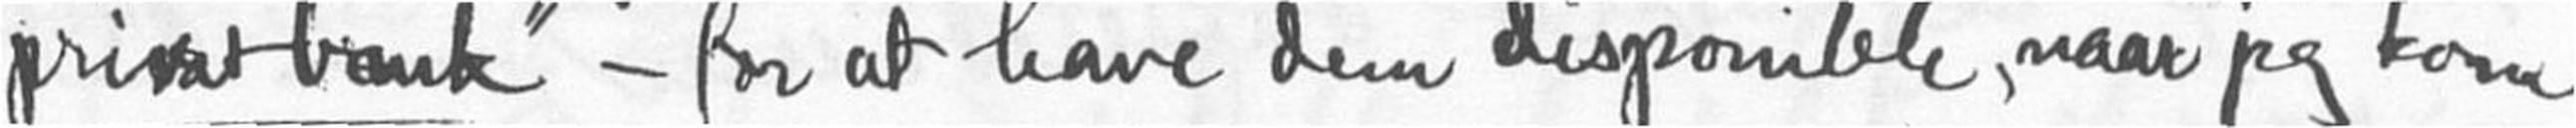privatbank" - for at have dem disponible, naar jeg kom
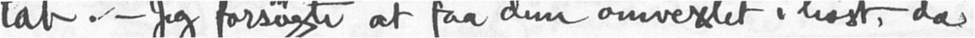tab. - Jeg forsøgte at faa dem omvexlet i høst, da
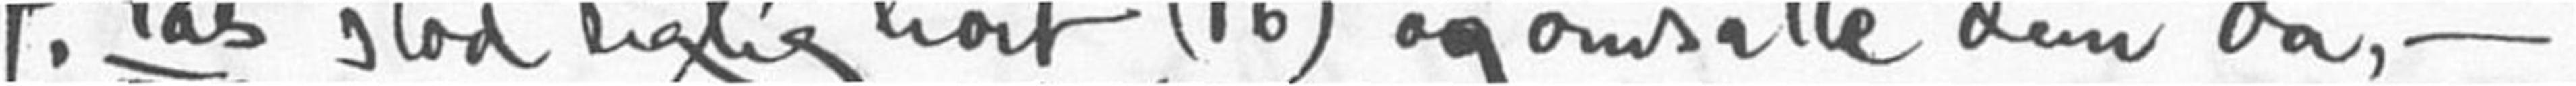P.tas stod rigtig høit (96) og omsatte dem da, -
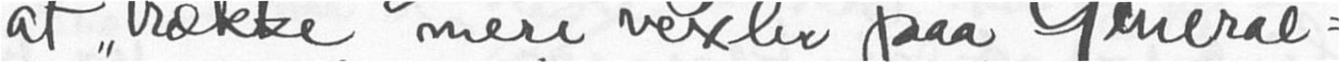at "trække mere vexler" paa General-
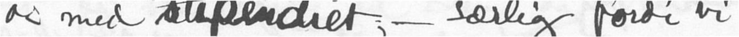os med stipendiet; - særlig fordi vi
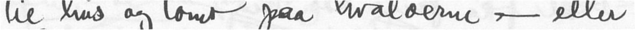til hus og tomt paa hvaløerne, - eller
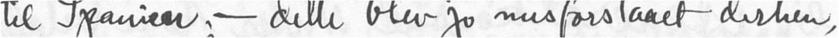til Spanien, - dette blev jo misforstaaet derhen,
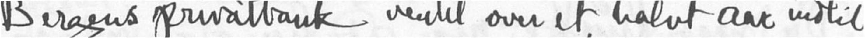Bergens privatbank ventet over et halvt aar indtil
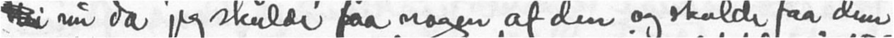nu da jeg skulde faa nogen af dem og skulde faa dem
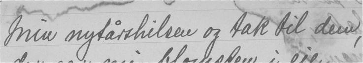Min nytårshilsen og tak til dem,
